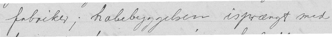fabriker; træbebyggelsen isprængt med
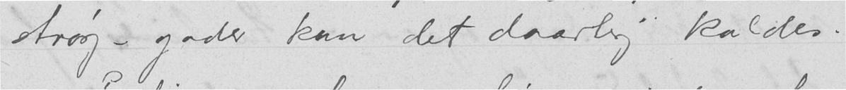strøg - gader kan det daarlig kaldes.
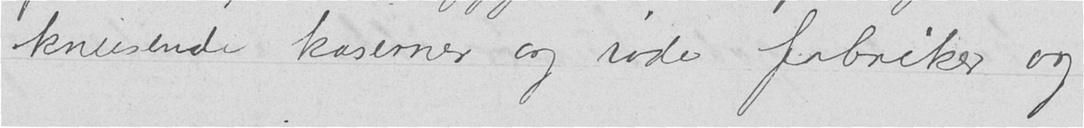kneisende kaserner og røde fabriker og
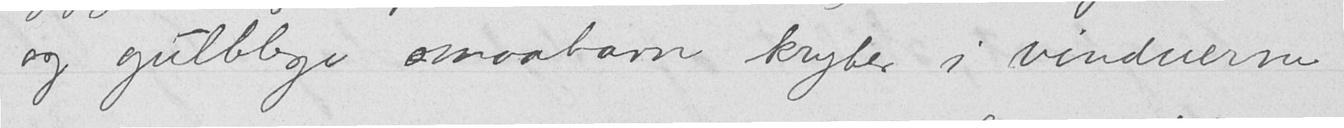og gulblege smaabarn kryber i vinduerne
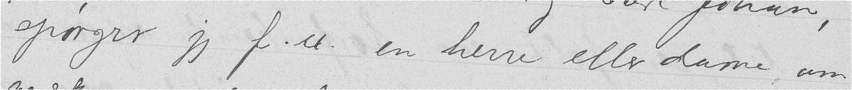spørger jeg f.ex. en herre eller dame om
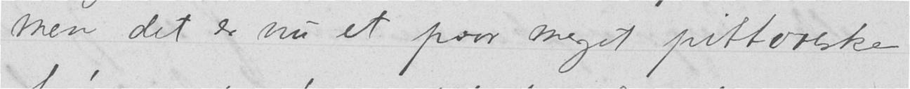men det er nu et par meget pittoreske
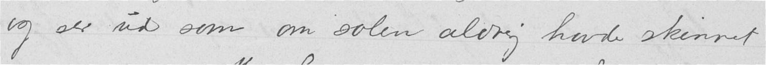og ser ud som om solen aldrig havde skinnet
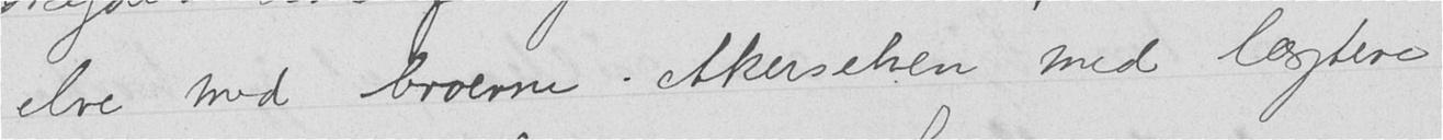elve med broerne. Akerselven med lægtere
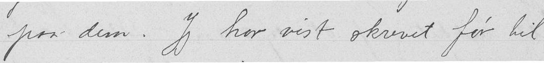paa dem. Jeg har vist skrevet før til
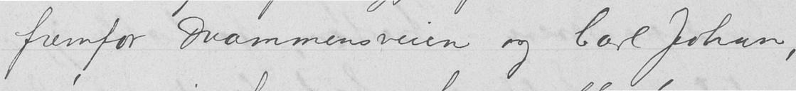fremfor Drammensveien og Carl Johan,
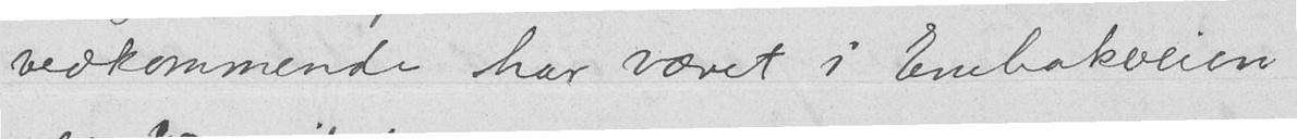vedkommende har været i Enebakveien
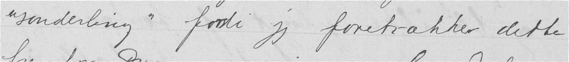«sonderling» fordi jeg foretrækker dette
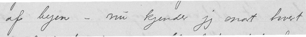af byen - nu kjender jeg snart hvert
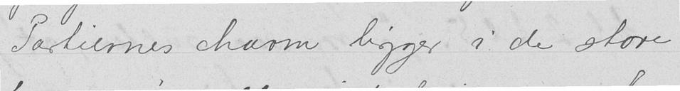Partiernes charme ligger i de store
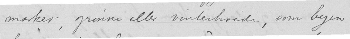marker, grønne eller vinterhvide, som byen
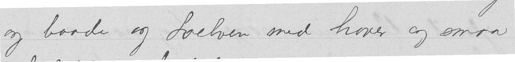og baade og Loelven med haver og smaa
